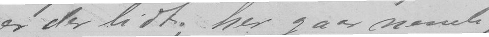er der lidt; her gaar nemlig
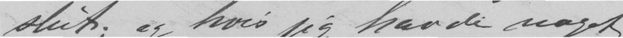slut; og hvis jeg havde noget
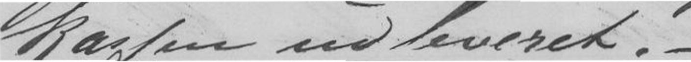kassen udleveret. -
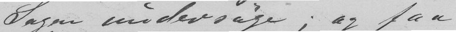Sagen undersøge; og faa
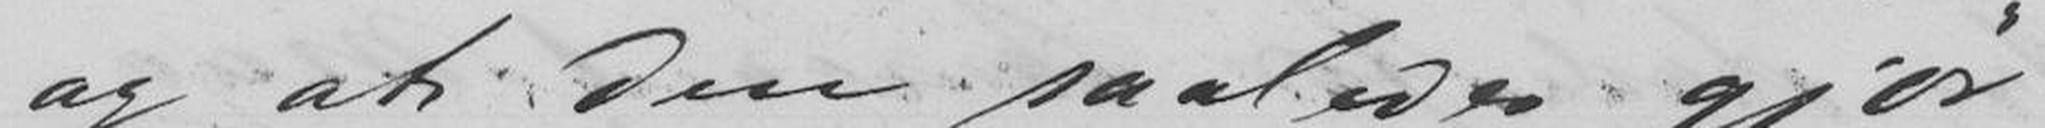og at den saaldes gjør
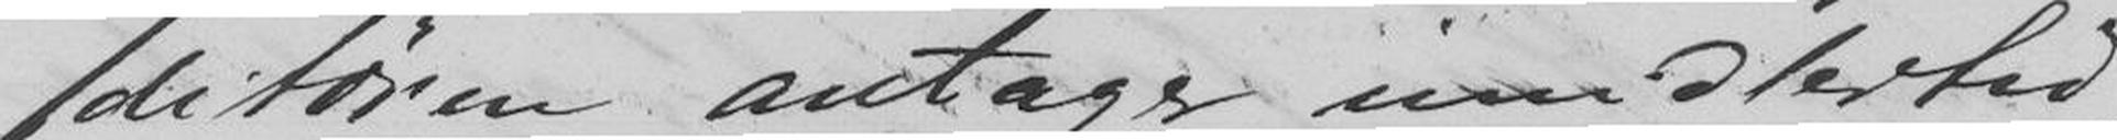ditøren antager imidlertid,
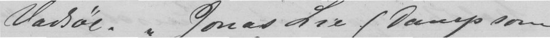Vadsøe. "Jonas Lie (Damp som
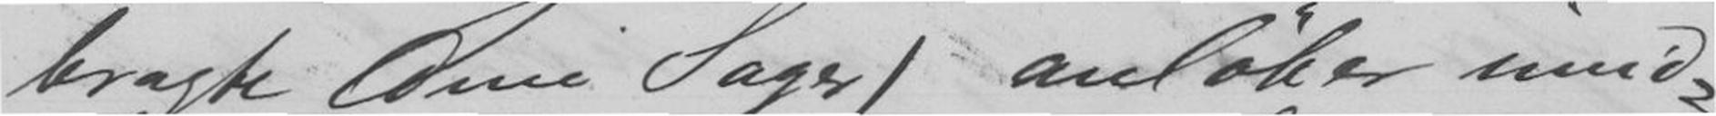bragte Dine Sager) anløber imid-
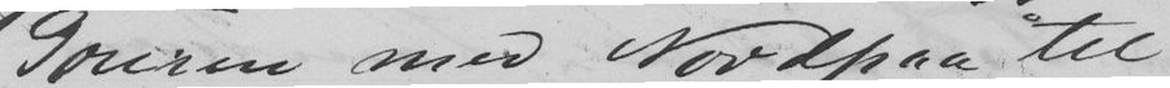Touren med Nordpaa til
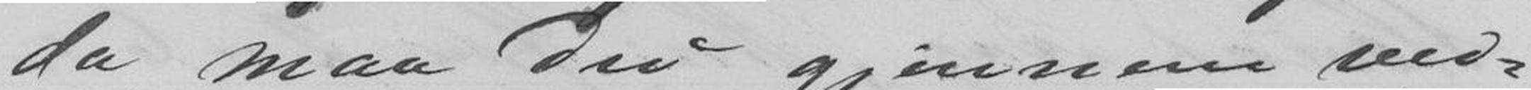da maa du gjennem ved-
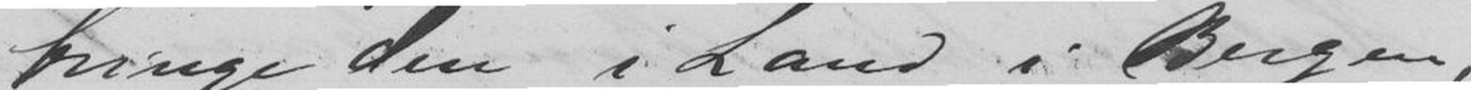bringe den iland i Bergen,
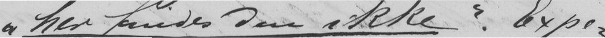her findes den ikke. Expe-
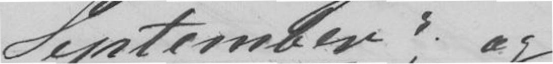September, og
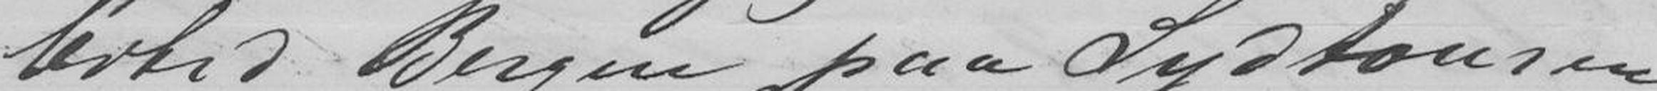lertid Bergen paa Sydtouren
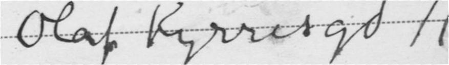Olaf Kyrresgd 11
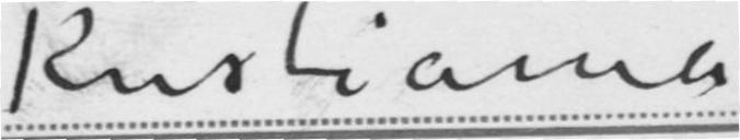Kristiania
